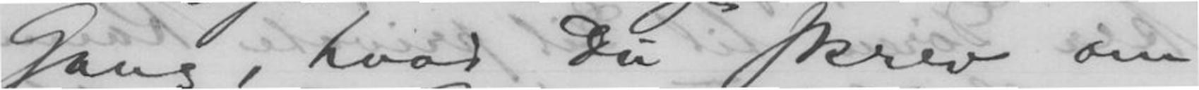Gang, hvad Du skrev om
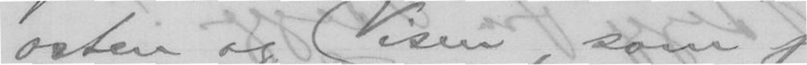osten og Visen, som jeg
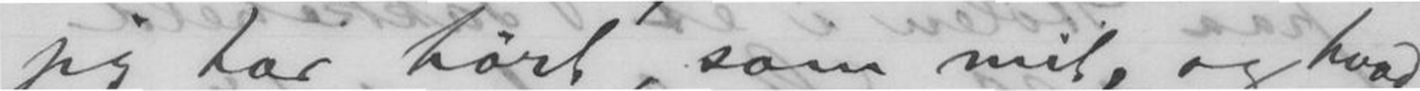jeg har hørt, som mit, og hvad
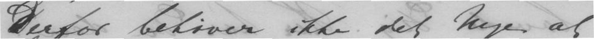Derfor behøver ikke det Nye at
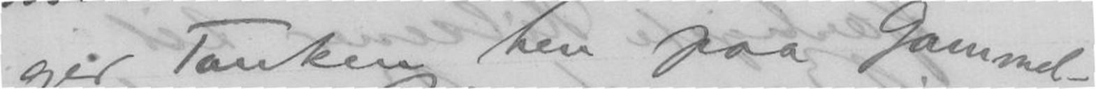ger Tanken hen paa Gammel-
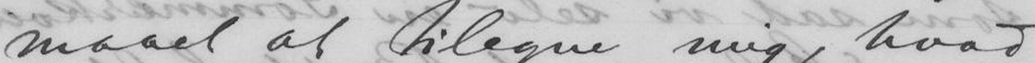maaet at tilegne mig, hvad
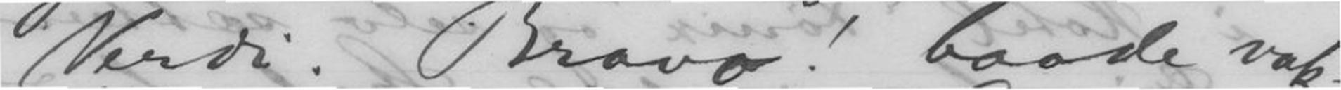Verdi. Bravo! baade vak-
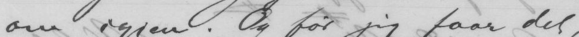om igjen. Og før jeg faar det,
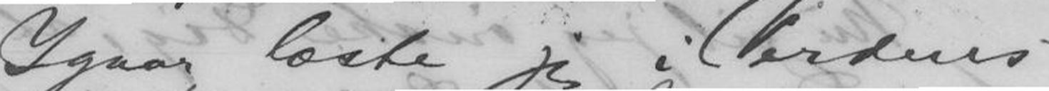Iggar læste jeg i Verdens
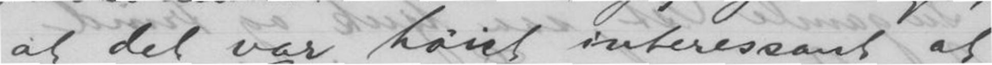at det var høist interessant at
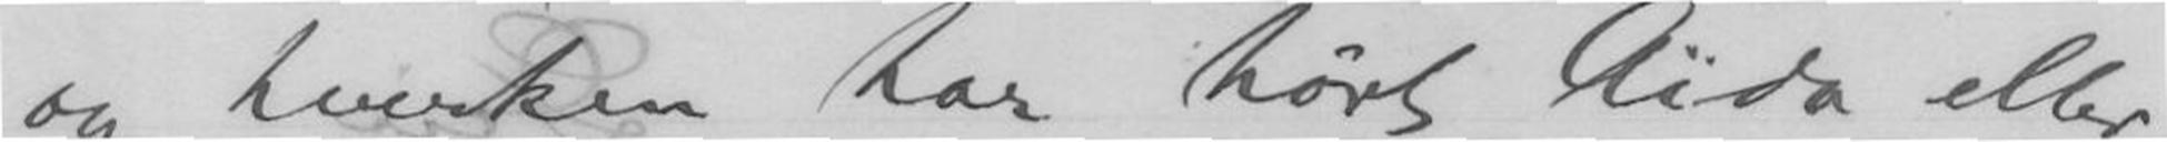og hverken har hørt Aïda eller
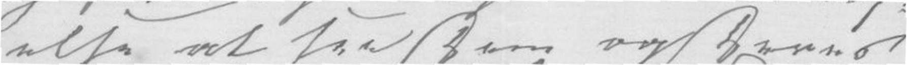else at see Dem og Deres
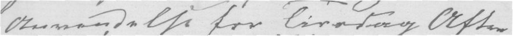Anvendelse for Tirsdag Aften
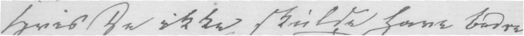Hvis De ikke skulde have bedre
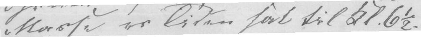Mosse er tiden sat til kl. 6 1/2.
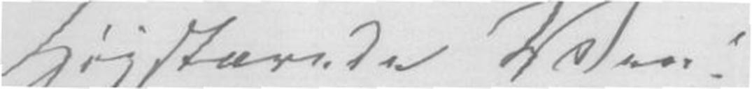Höjstærede Ven!
